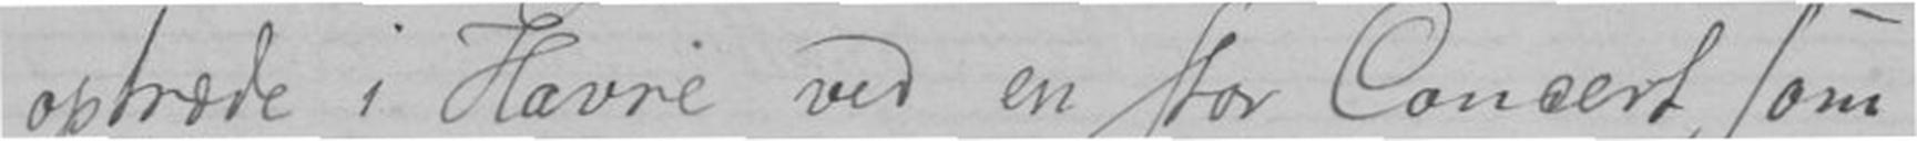optræde i Havre ved en stor Concert, som
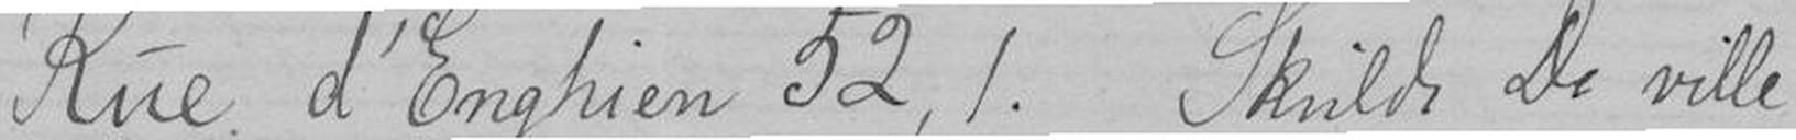Rue d'Enghien 52,1. Skulde De ville
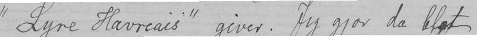"Lyre Havreais" giver. Jeg gjør da blot
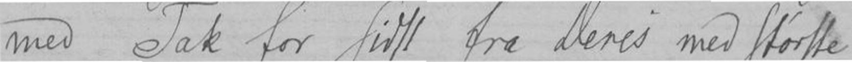med Tak for sidst fra Deres med største
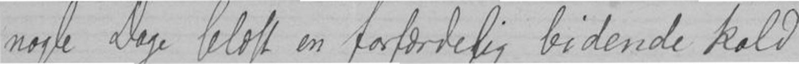nogle Dage blæst en forfærdelig bidende kold
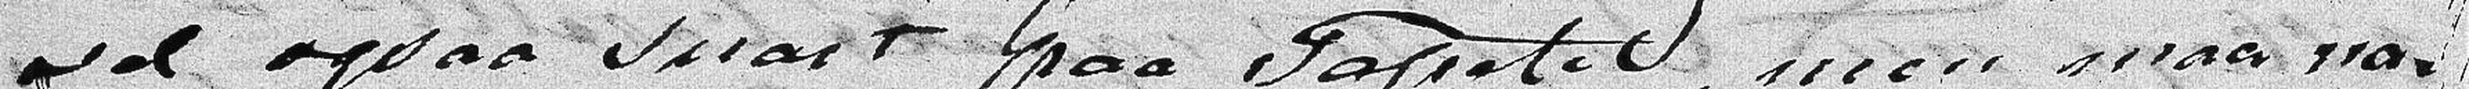vel ogsaa snart paa Tapetet, men maa na-
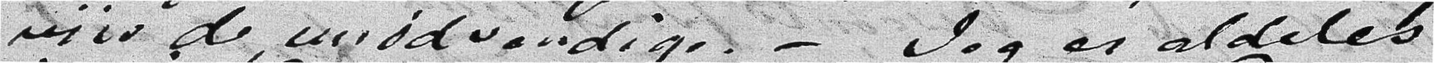viis de unødvendige. - Jeg er aldeles
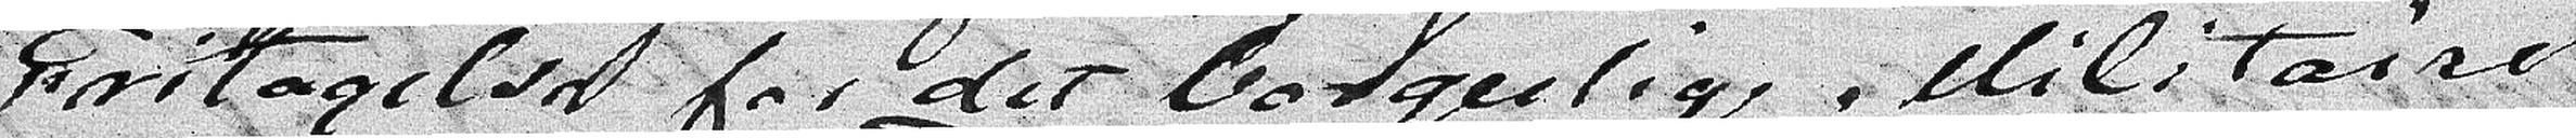Fritagelse for det borgerlige Militaire
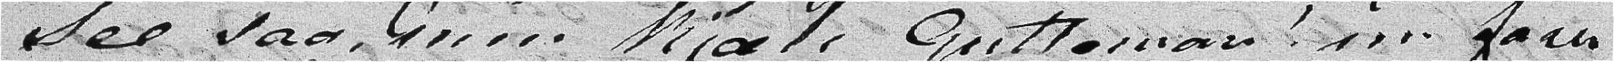See saa, min kjære Gutteman! nu faaer
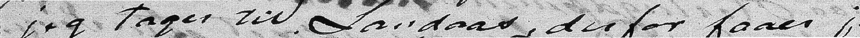jeg tager til Landaas, derfor faaer jeg
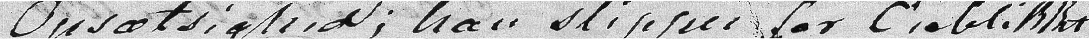Opsætsighed; han slipper for Øieblikket
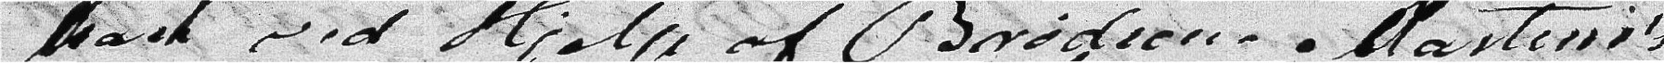han ved Hjelp af Brøderne Martens's
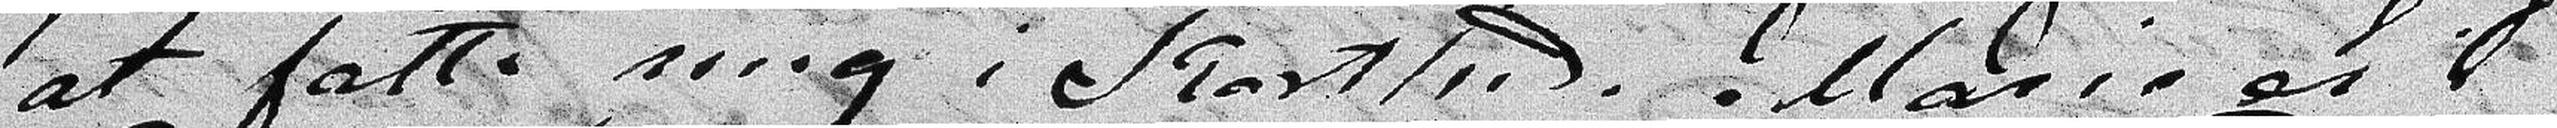at fatte mig i Korthed. Marie er i
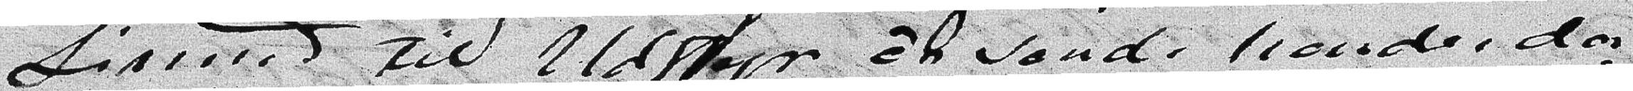Linned til Udstyr en sende hende, der
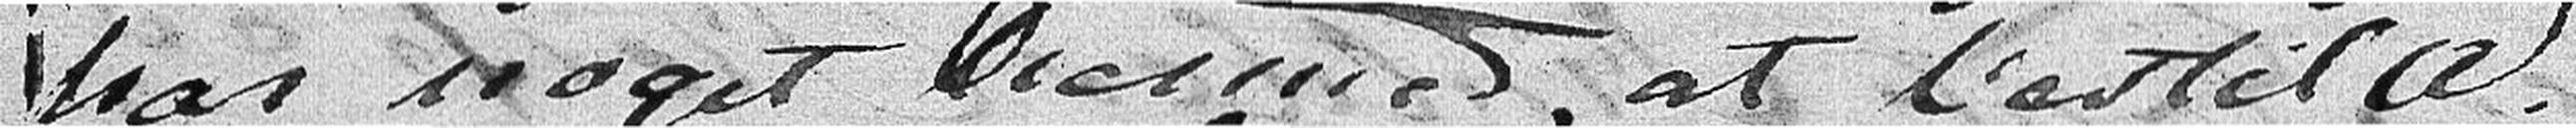har noget hermed at bestille.
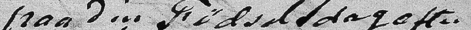paa sin Fødselsdag efter
